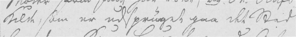Kilde, som er udsprunget paa det Sted
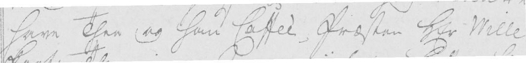have Thee og han Caffee - Præsten Hr Wille
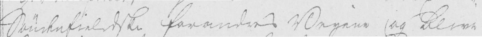Søndenfieldske forandres Veyene og bliver
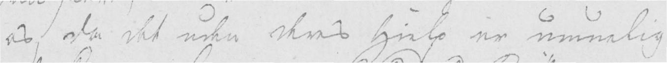os, da det uden deres Hielp er umuelig
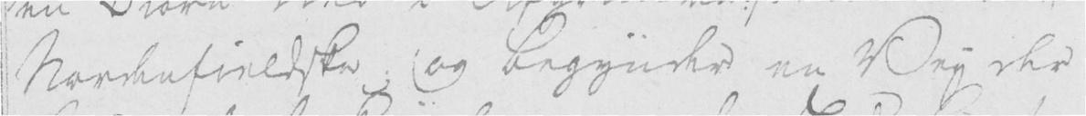Nordenfieldske, og begynder en Vej der
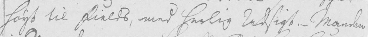høyt til Fields, med herlig Udsigt. - Manden
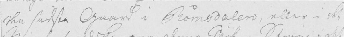den sidste Gaard i Romsdalen, eller i det
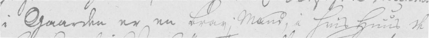i Gaarden er en brav Mand, i hvis Huus de
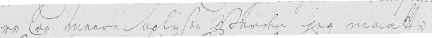re og meere oplyste Verden jeg maatte
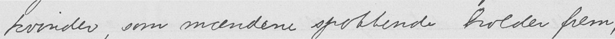kvinder, som mændene spottende holder frem,
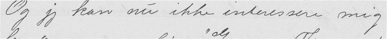Og jeg kan nu ikke interessere mig
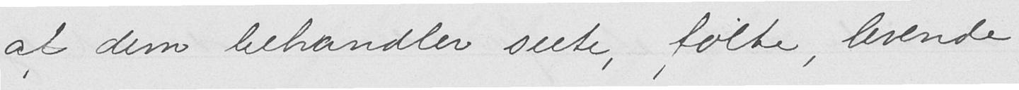af dem behandler seete, følte, levende
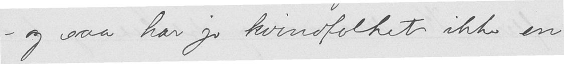- og saa har jo kvindfolket ikke en
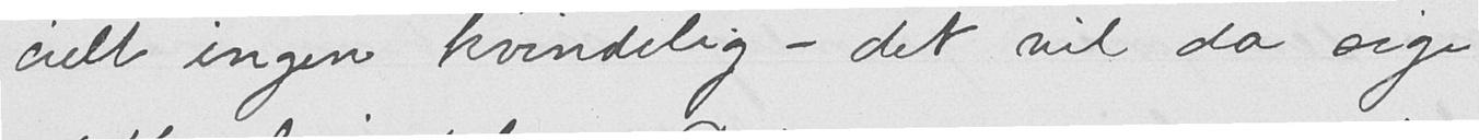cielt ingen kvindelig - det vil da sige
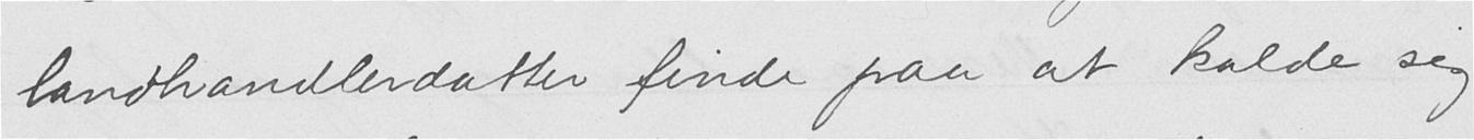landhandlerdatter finde paa at kalde sig
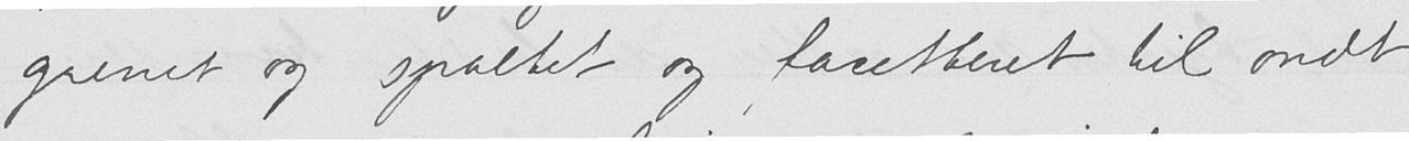grenet og spaltet og facetteret til ondt
